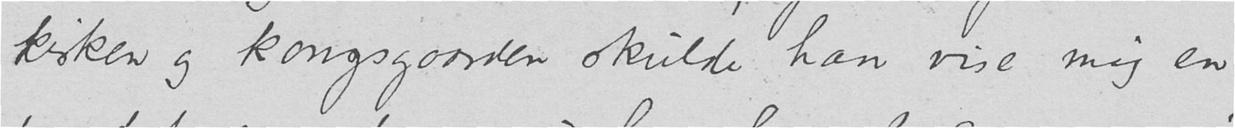kirken og kongsgaarden skulde han vise mig en
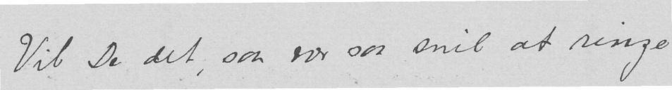Vil De det, saa vær saa snil at ringe
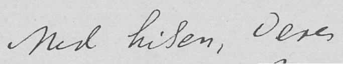Med hilsen, Deres
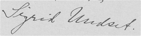Sigrid Undset.
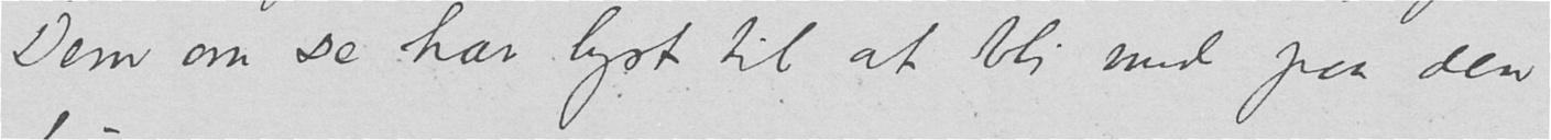Dem om De har lyst til at bli med paa den
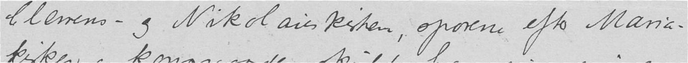Clemens – og Nikolauskirken, sporene efter Maria-
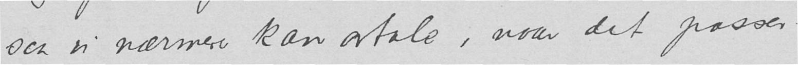saa vi nærmere kan avtale, naar det passer.
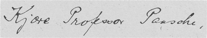Kjære Professor Paasche,
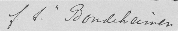f.t. "Bondeheimen"
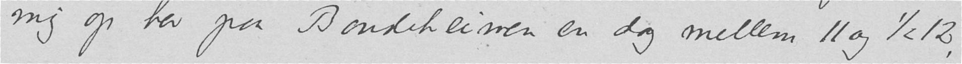mig op her paa Bondeheimen en dag mellem 11 og 1/2 12,
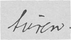turen.
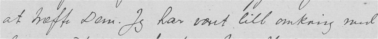at træffe Dem. Jeg har været litt omkring med
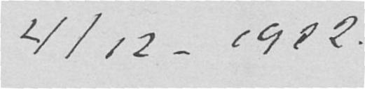4/12-1922.
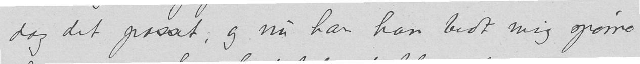dag det passet, og nu har han bedt mig spørre
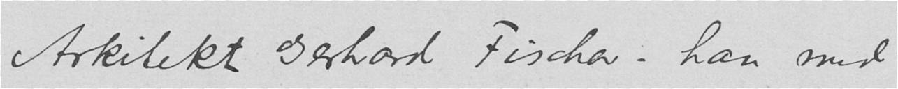Arkitekt Gerhard Fischer - han med
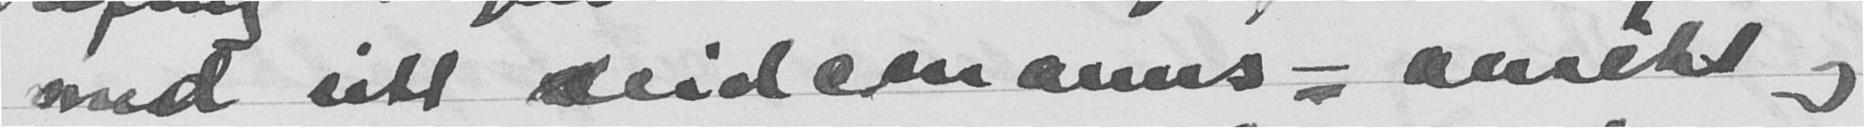med sitt seidemanns-ansikt og
